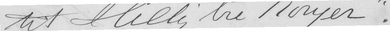til Hellig tre Konger
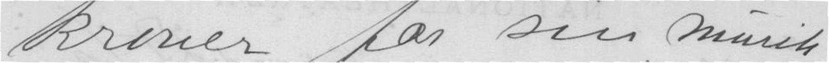kroner for sin musik
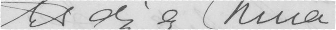til dig og Nina
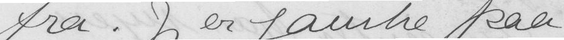fra. Jeg er ganske paa
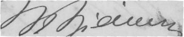B. Bjørnson
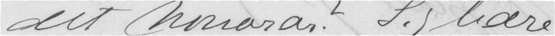det honorar? Sig bare
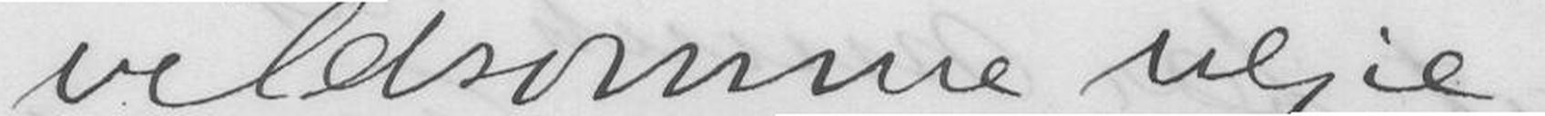vildsomme veje
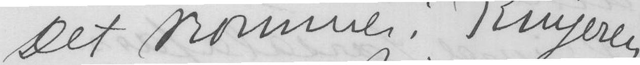Det kommer i Ringeren
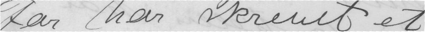Far har skrevet et
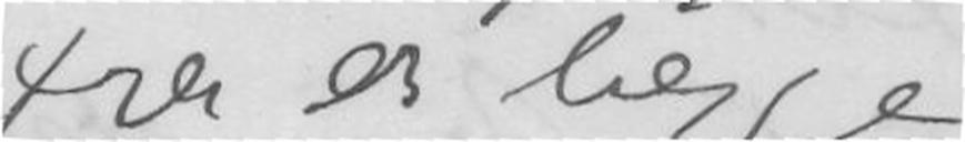fra os begge.
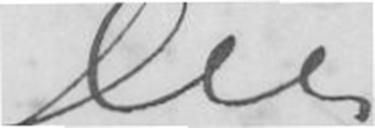Din
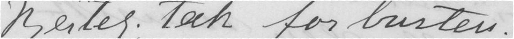Hjertelig Tak for busten.
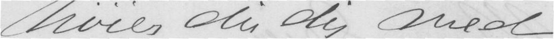Nøier du dig med
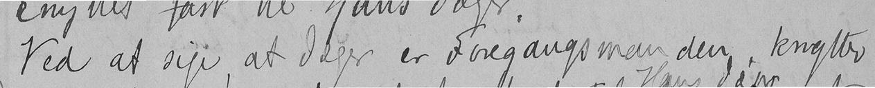Ved at sige, at Jæger er Foregangsmanden, knytter
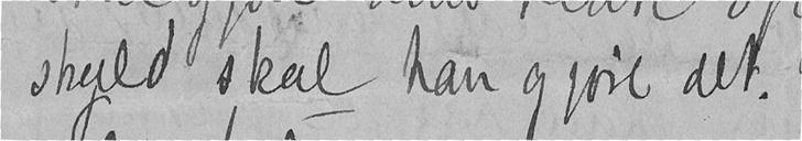skyld skal han gjøre det.
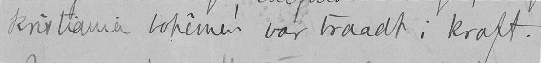Kristiania bohêmen var traadt i kraft.
